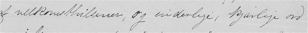af velkomsthilsener, og inderlige, kjærlige ord
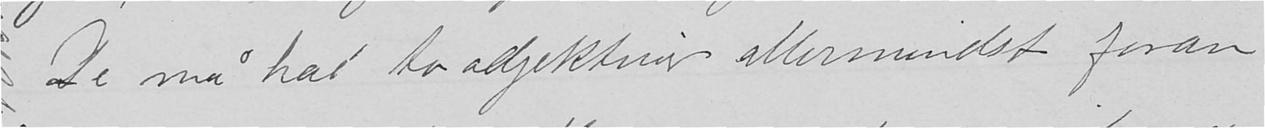De må ha to adjektiver allermindst foran
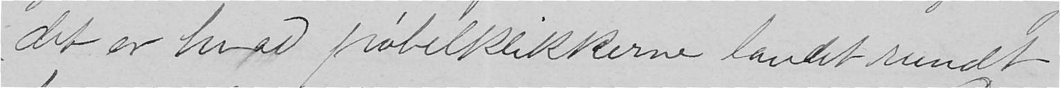det er hvad pøbelklikkerne landet rundt
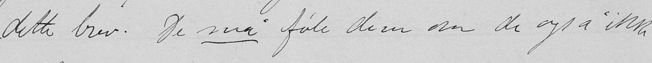dette brev. De må føle dem om de også ikke
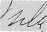hele
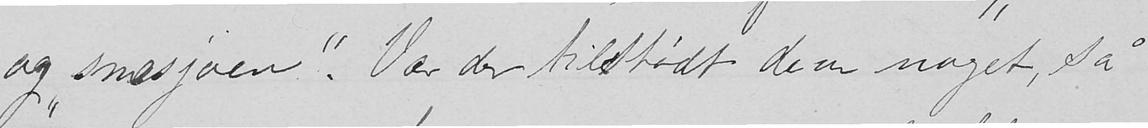og "snæsjøen". Var der tilstødt dem noget, så
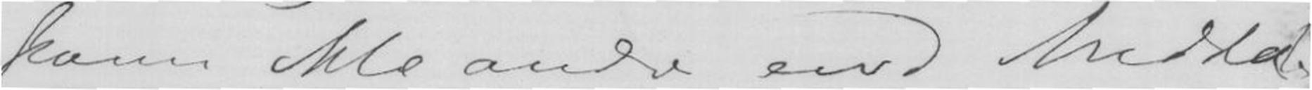kome ikke andre end Meddals-
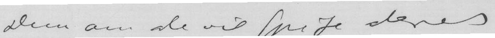Dem om de vil spise Deres
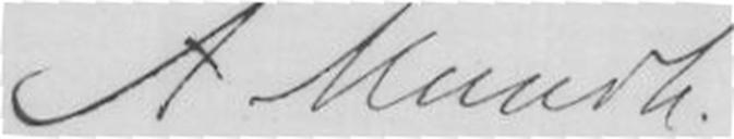A. Munch.
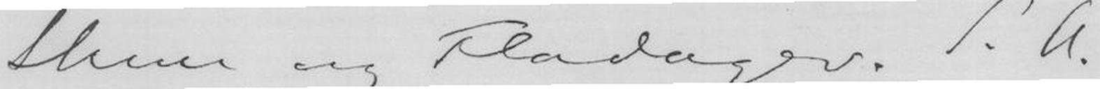thun og Fladager. S. A.
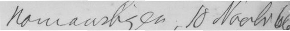Homansbyen, 18 Novbr 66
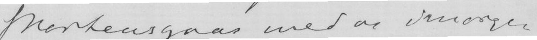Mortensgaas med os imorgen
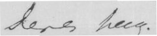Deres heng.
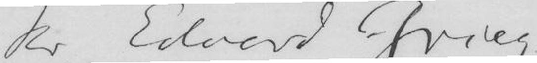Hr. Edvard Grieg
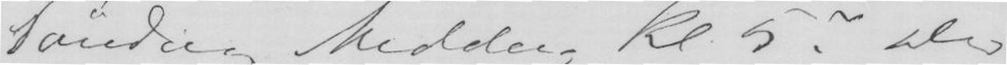Søndag Middag Kl. 5. Det
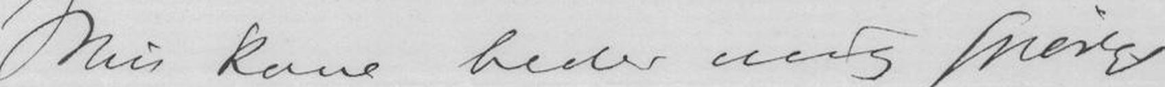Min kone beder mig spørge
